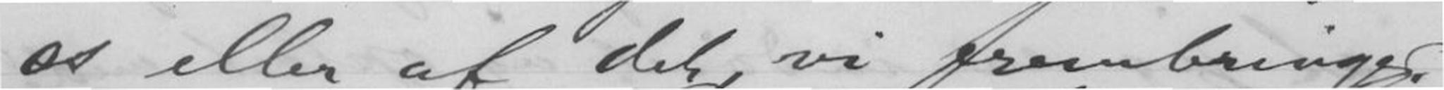os eller af det, vi frembringer.
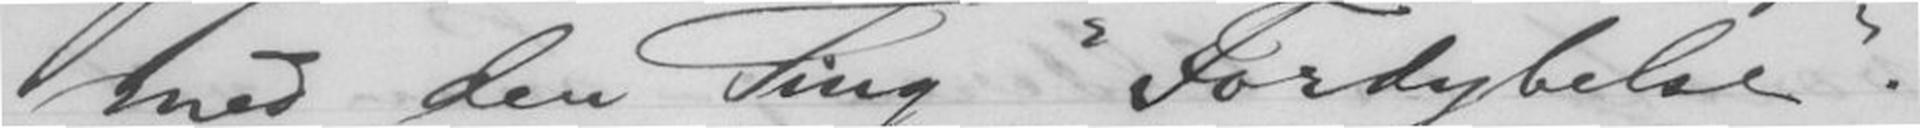med den Ting "Fordybelse".
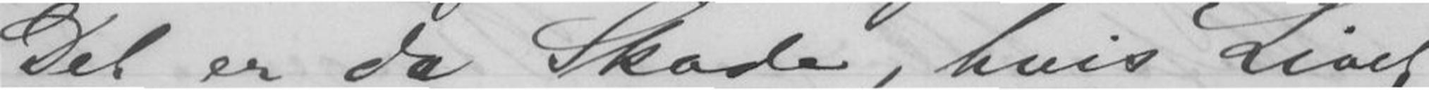Det er da Skade, hvis Livet
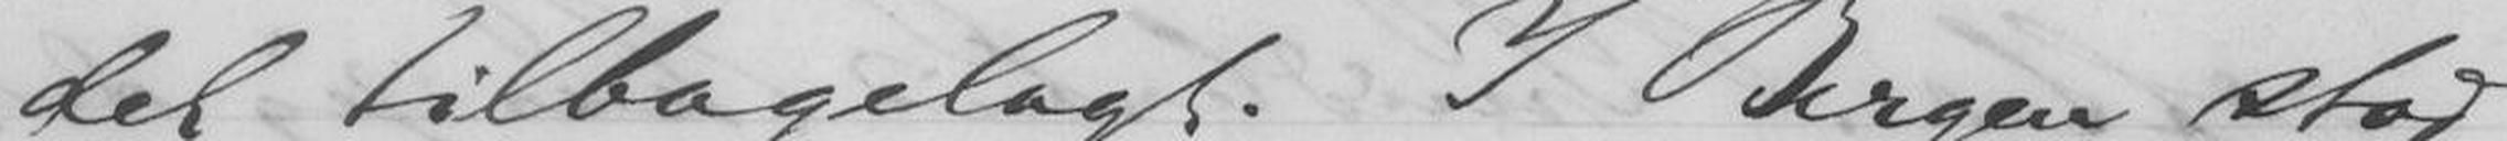det tilbagelagt. I Bergen stod
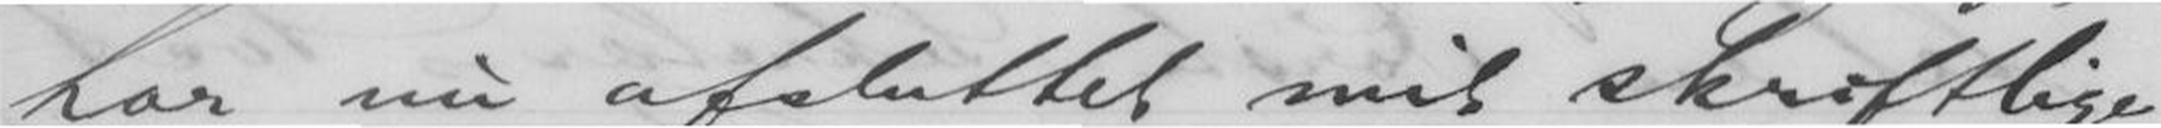har nu afsluttet mit skriftlige
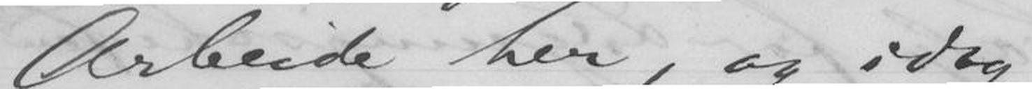Arbeide her, og idag
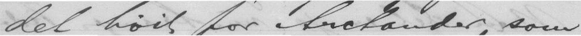det høit for Arctander, som
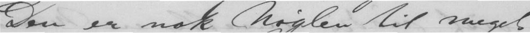Den er nok Nøglen til meget
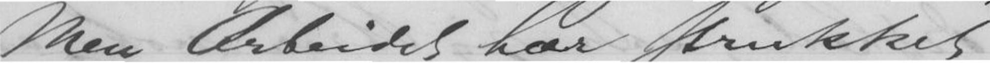Men Arbeidet har strukket
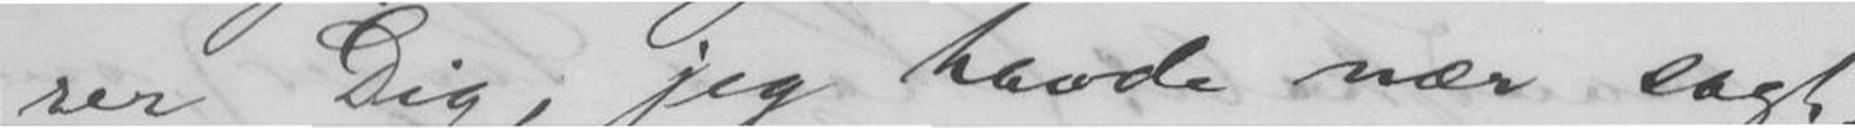rer Dig, jeg havde nær sagt,
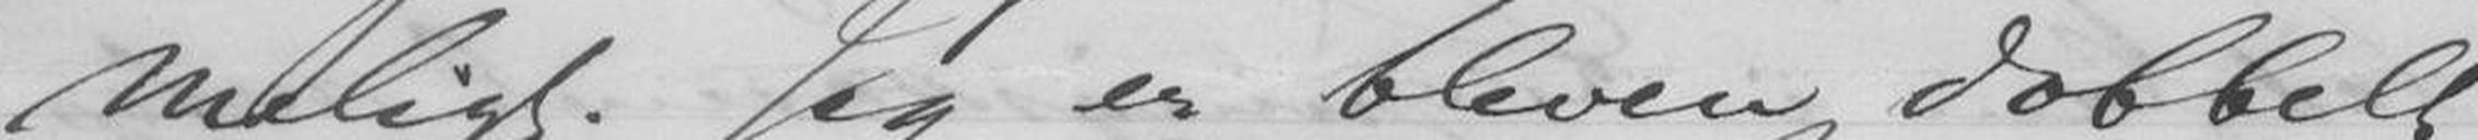meligt. Jeg er bleven dobbelt
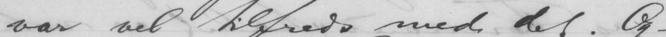var vel tilfreds med det. Og-
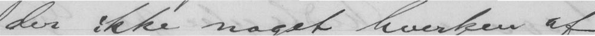der ikke noget hverken af
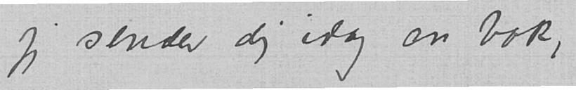jeg sender dig idag en bok,
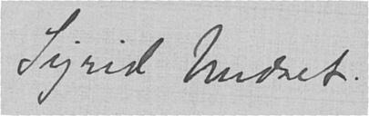Sigrid Undset.
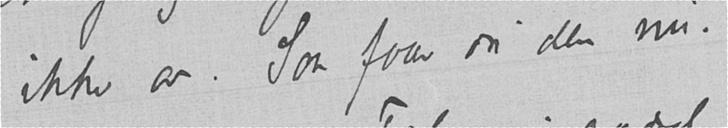ikke av. Saa faar du den nu.
